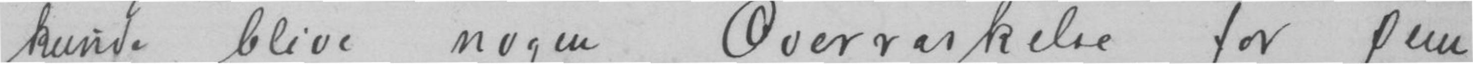kunde blive nogen Overraskelse for Dem,
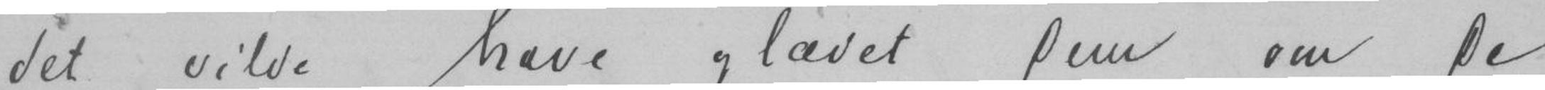det vilde have glædet Dem om De
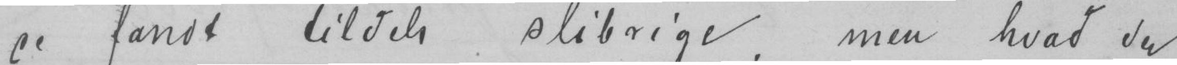de fandt tildels slibrige, men hvad det
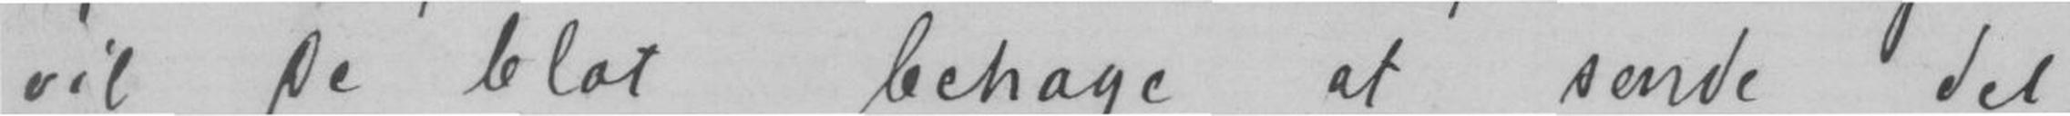vil De blot behage at sende det
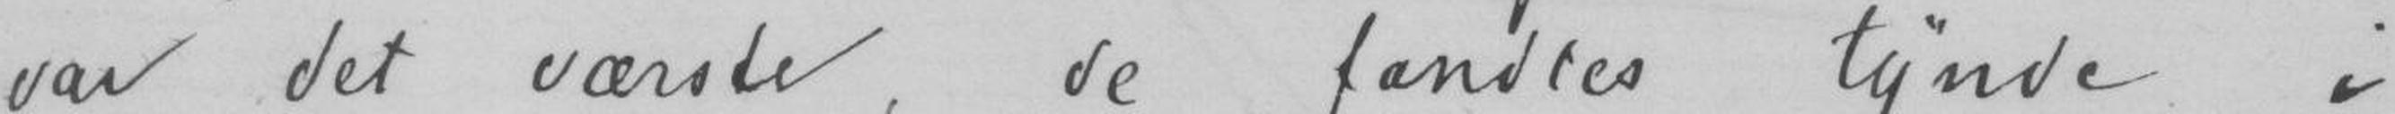var det værste, de fandtes tynde i
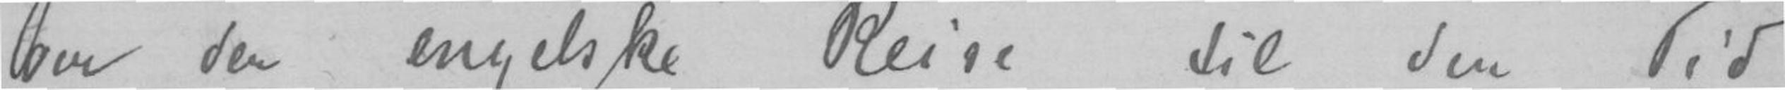om den engelske Reise til den Tid
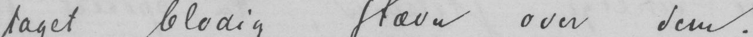taget blodig Hævn over dem.
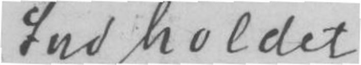Indholdet.
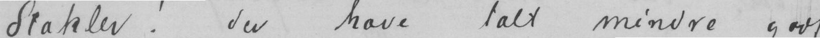Stakler!, der have talt mindre godt
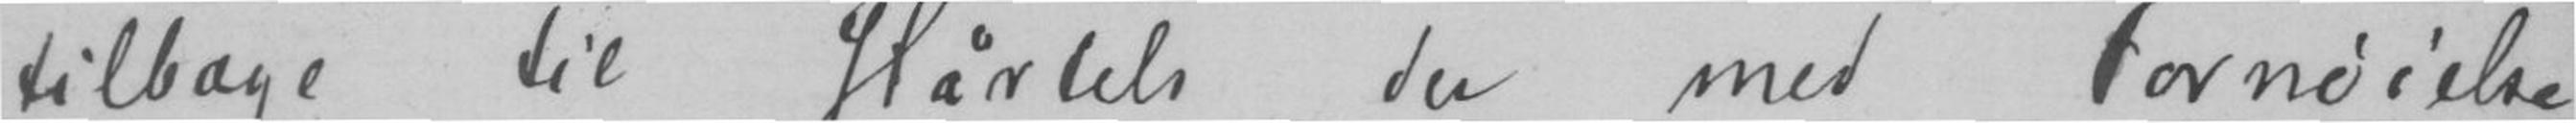tilbage til Hårtels, du med Fornøielse
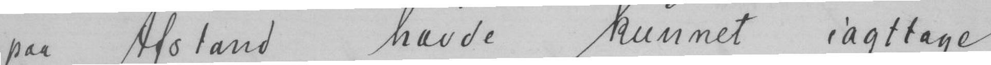paa Afstand havde kunnet iagttage
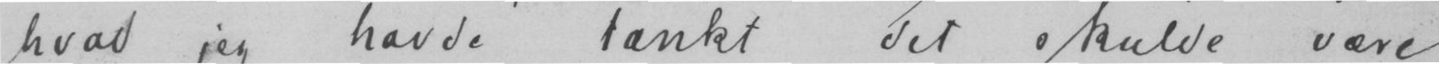hvad jeg havde tænkt det skulde være,
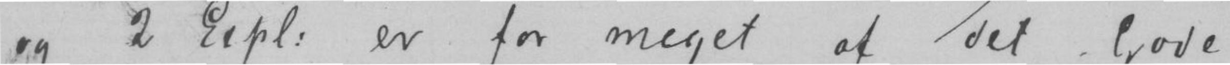og 2 Expl: er for meget af det Gode,
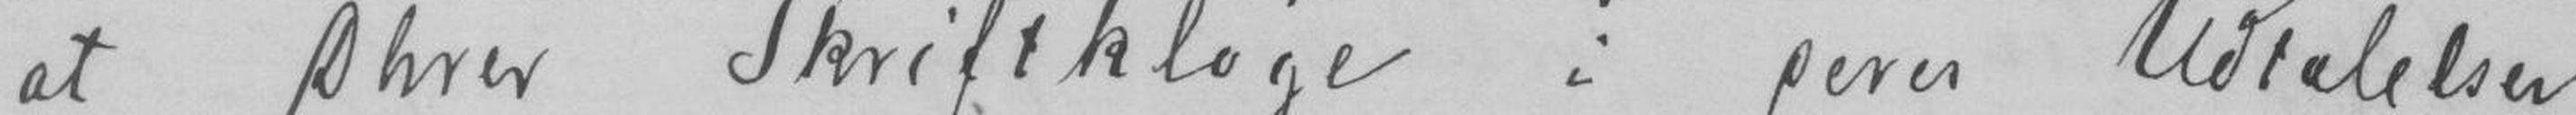at Dhrer Skriftklage i Deres Udtalelser
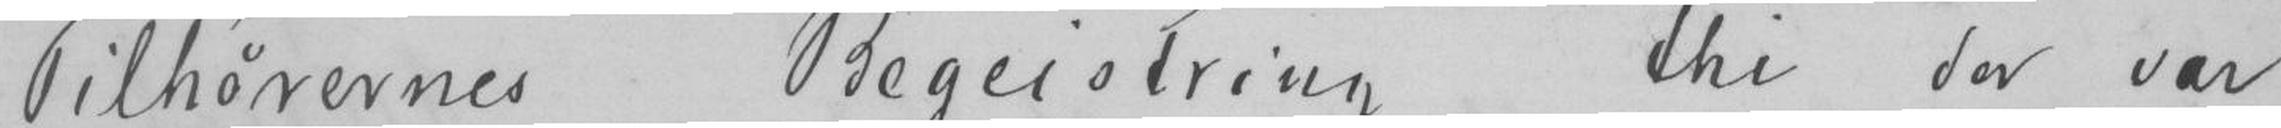Tilhørernes Begeistring, thi der var
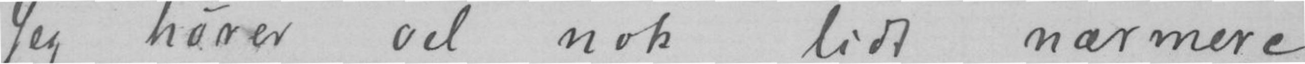Jeg hører vel nok lidt nærmere
